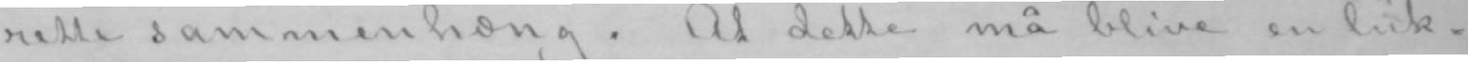rette sammenhæng. At dette må blive en luk-
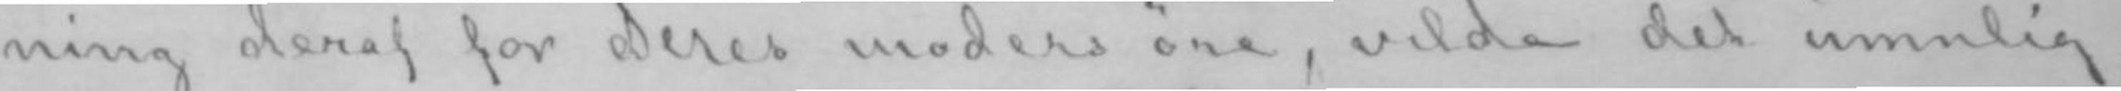ning deraf for Deres moders øre, vilde det umulig-
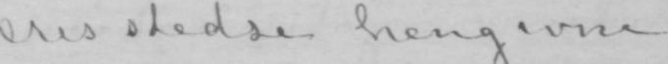Deres stedse hengivne
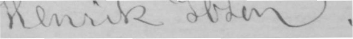Henrik Ibsen.
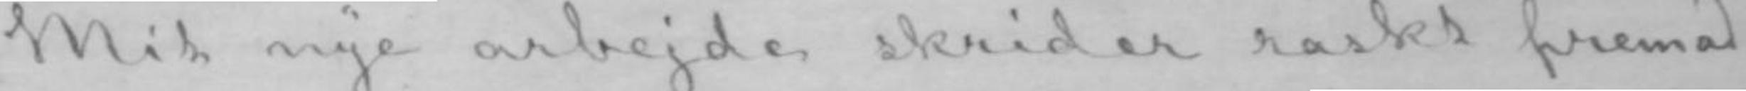Mit nye arbejde skrider raskt fremad.
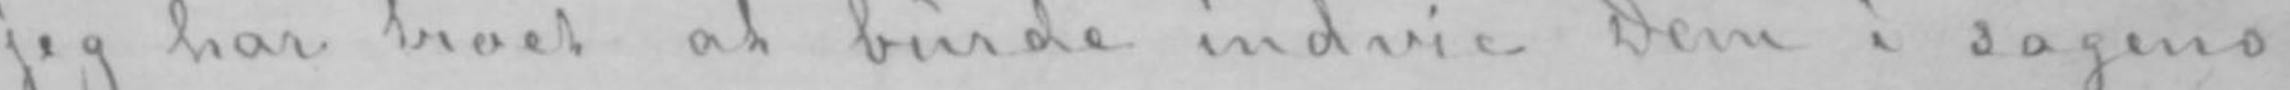jeg har troet at burde indvie Dem i sagens
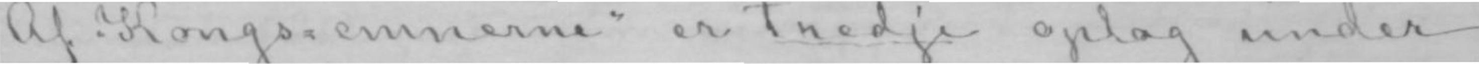Af «Kongs-emnerne» er Tredje oplag under
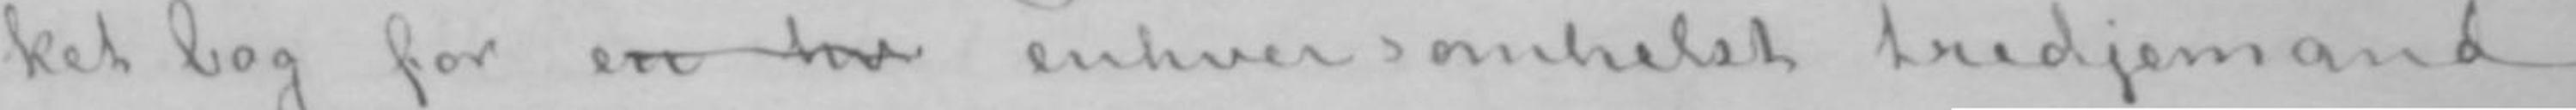ket bog for en hv enhver somhelst tredjemand
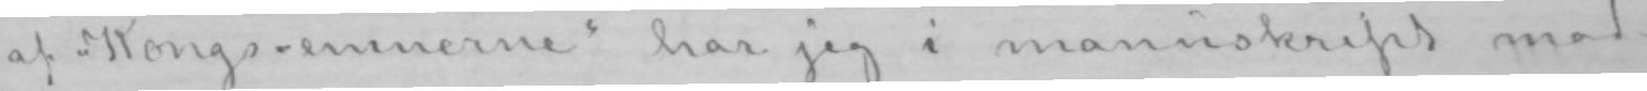af «Kongs-emnerne» har jeg i manuskript mod-
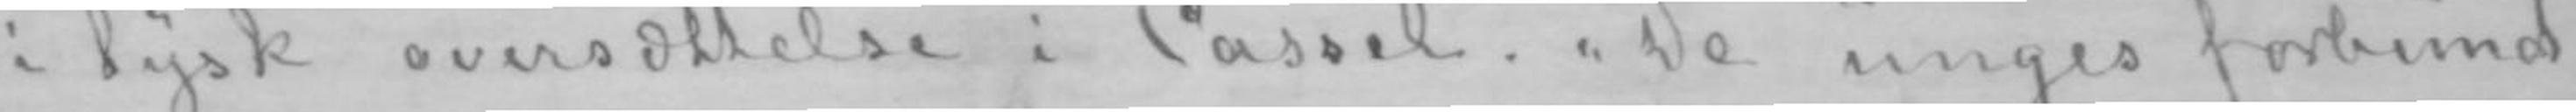i tysk oversættelse i Cassel. «De unges forbund»
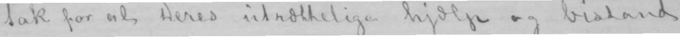tak for al Deres utrættelige hjælp og bistand!
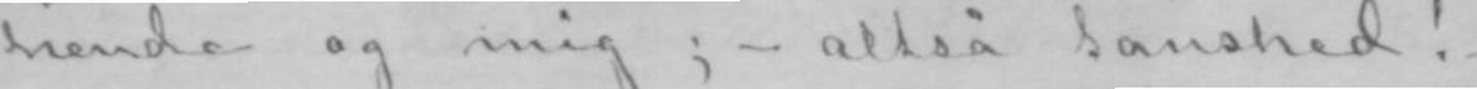hende og mig;- altså taushed!-
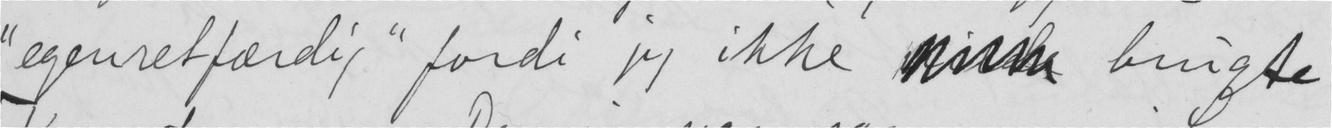"egenretfærdig" fordi jeg ikke  brugte
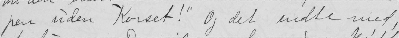pen uden Korset!" Og det endte med,
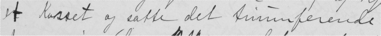et Korset og satte det triumferende
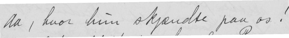Aa, hvor hun skjændte paa os!
